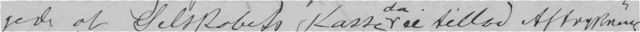gede at Selskabets Kasse ei tillod Aftrykning
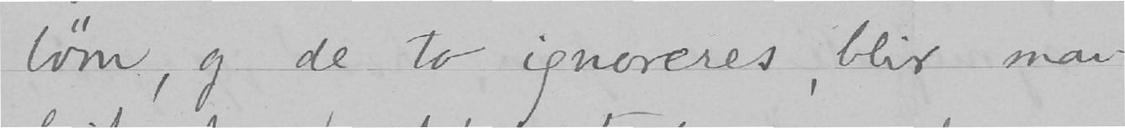børn, og de to ignoreres, blir man
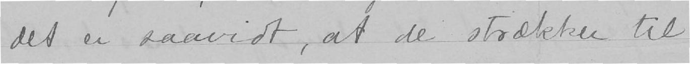det er saavidt, at de strækker til.
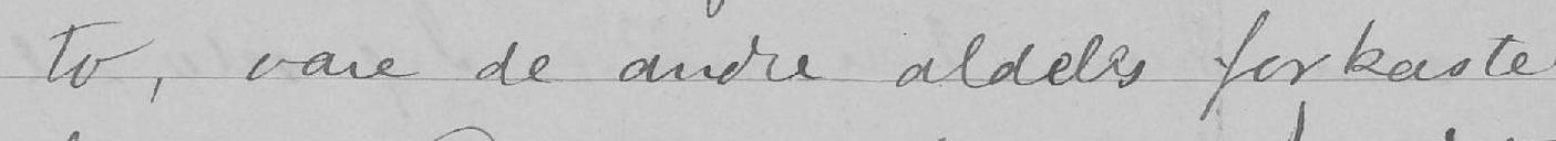to, vare de andre aldeles forkaste-
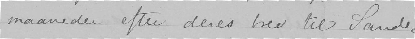maaneder efter deres brev til Sande-
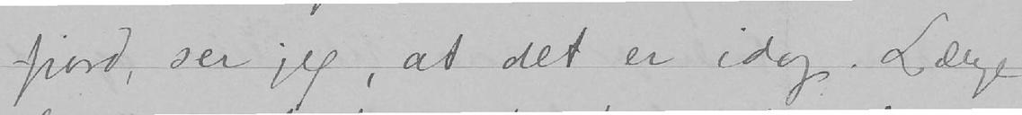fiord, ser jeg, at det er idag. Længe
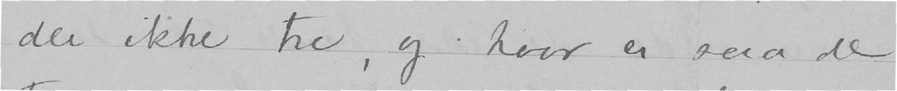der ikke tre, og hvor er saa de
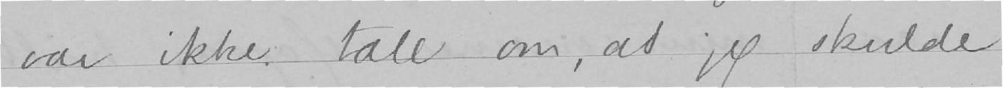var ikke tale om, at jeg skulde
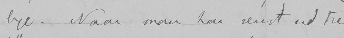lige. Naar man har sendt ud tre
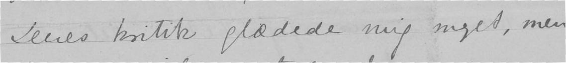Deres kritik glædede mig meget, men
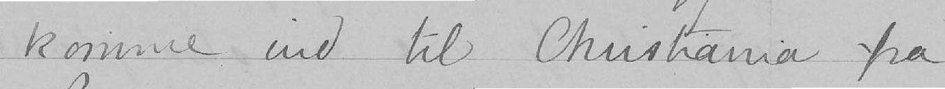komme ind til Christiania fra
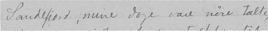Sandefiord, mine dage vare nøie talte,
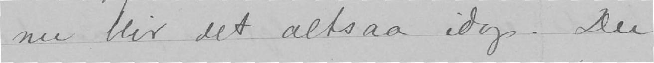nu blir det altsaa idag. Der
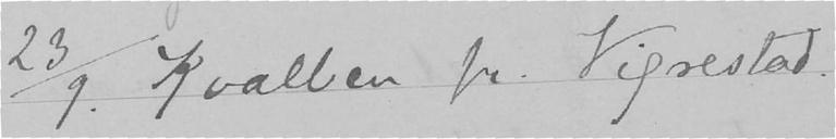23/9. Kvalben pr. Vigrestad.
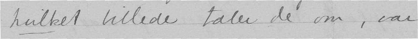hvilket billede taler de om, var
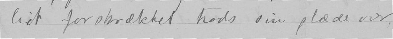lidt forskrækket trods sin glæde over,
